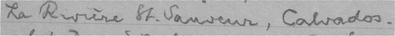La Rivière St. Sauveur, Calvados.
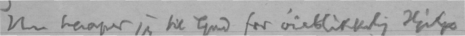Nu haaper jeg til Gud for øieblikkelig Hjelp
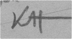KH
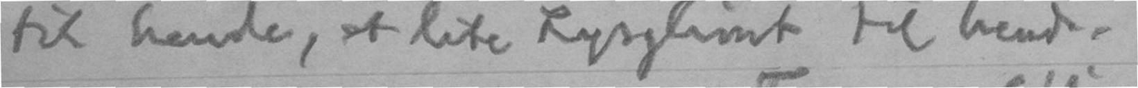til hende, et lite Lysglimt til hende.
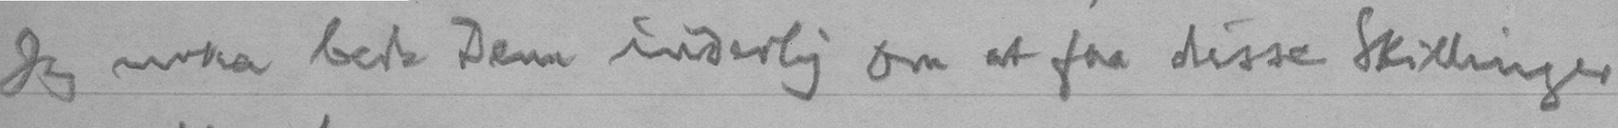Jeg maa bede Dem inderlig om at faa disse Skillinger
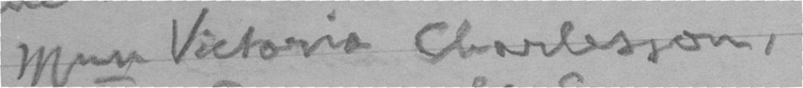Mme Victoria Charlesson,
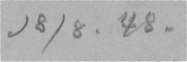18/8. 48.
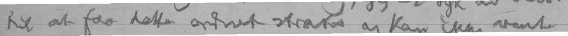til at faa dette ordnet straks og kan ikke vente
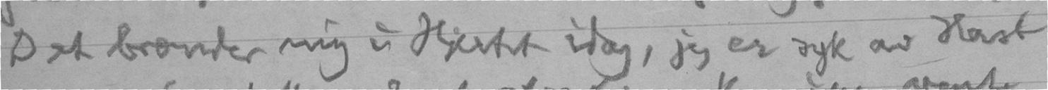Det brænder mig i Hjertet idag, jeg er syk av Hast
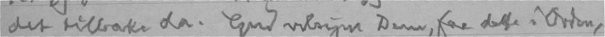det tilbake da. Gud velsigne Dem, faa dette i Orden,
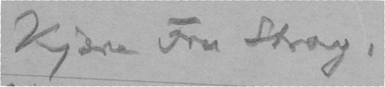Kjære Fru Stray,
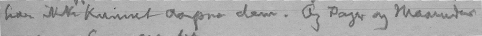har ikke kunnet aapne dem. Og Dager og Maaneder
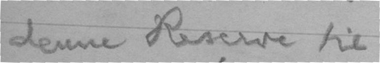denne Reserve til
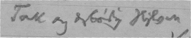Tak og ærbødig Hilsen
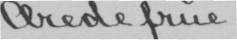Ærede frue,
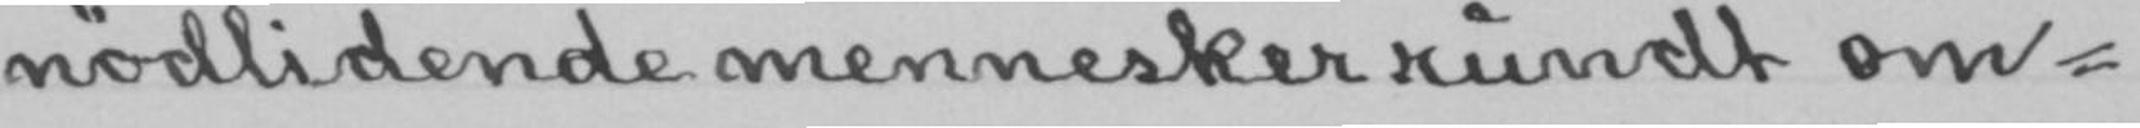nødlidende mennesker rundt om-
